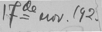17de nov. 1925
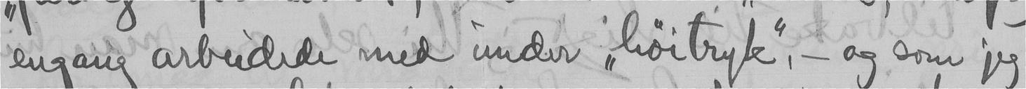engang arbuidede med under "høitryk", - og som jeg
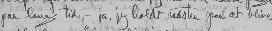paa lang tid; - ja, jeg holdt næsten paa at blive
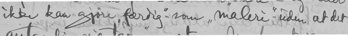ikke kan gjøre "færdig" - som "maleri", - uden at det
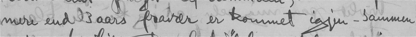mere end 3 aars fravær er kommet igjen - sammen
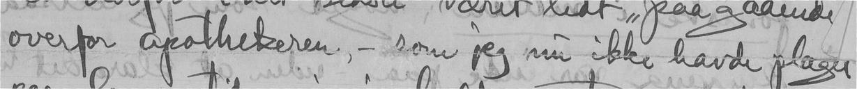overfor apothekeren, - som jeg nu ikke havde plaget
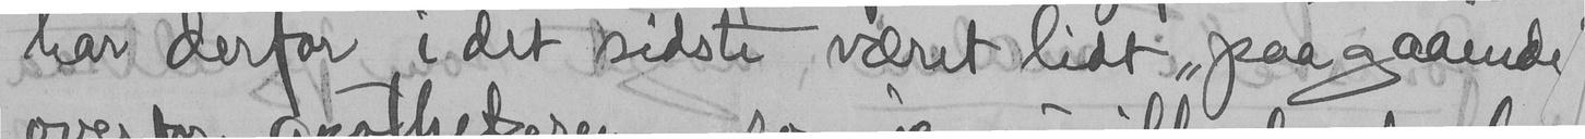har derfor i det sidste været lidt "paagaaende"
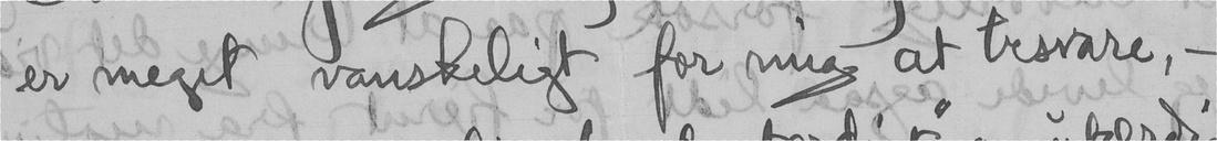er meget vanskeligt for mig at besvare, -
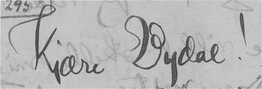Kjære Bydal!
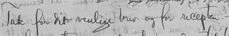Tak for dit venlige brev og for recepten! -
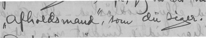"afholdsmand", som du siger.
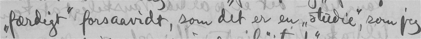"færdigt" forsaavidt, som det er en "studie", som jeg
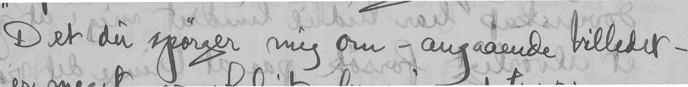Det du spørger mig om - angaaende billeder -
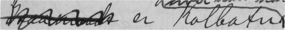er Kolbotn
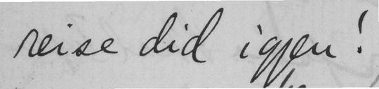reise did igjen!
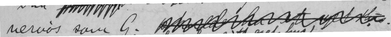nervøs som G.
